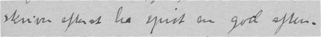skriver efterat ha spist en god aftens.
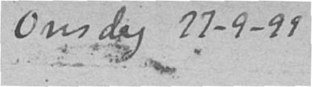Onsdag 27-9-99
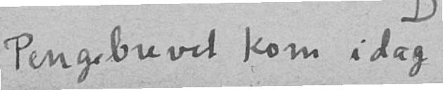Pengebrevet kom i dag
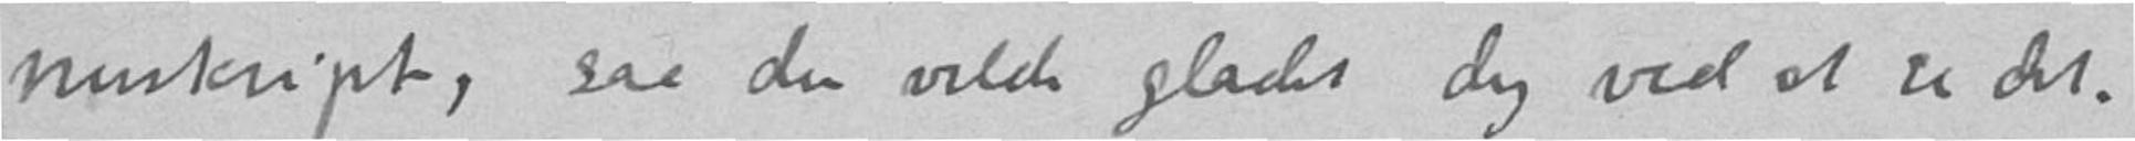nuskript, saa du vilde glædet dig ved at se det.
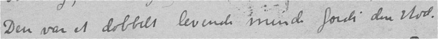Den var et dobbelt levende minde fordi den stod.
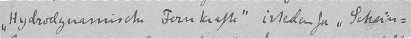"Hydrodynamische Fernkrafte" istedenfor "Schein-
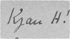Kjære H!
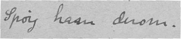Spørg ham derom.
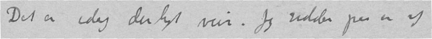Det er idag deiligt veir. Jeg sidder paa en af
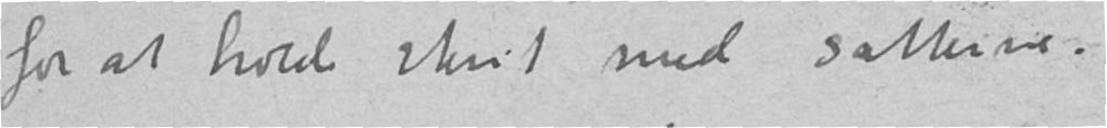for at holde trit med sætterne.
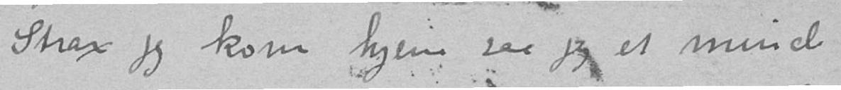Strax jeg kom hjem saa jeg et minde
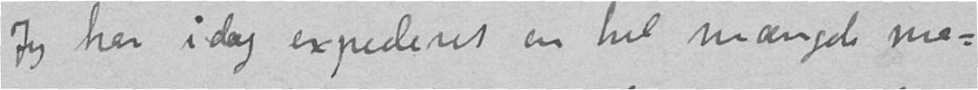Jeg har i dag expederet en hel mængde ma-
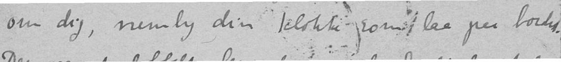om dig, nemlig din klokke som laa paa bordet.
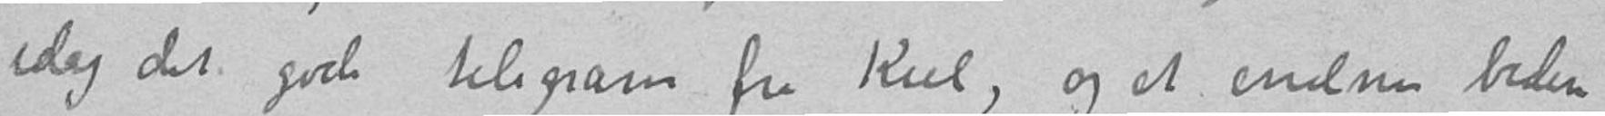idag det gode telegram fra Kiel, og et endnu bedre
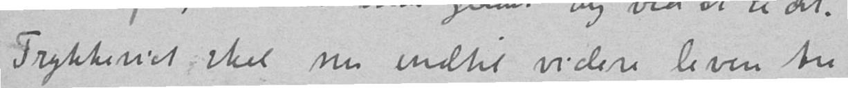Trykkeriet skal nu indtil videre levere tre
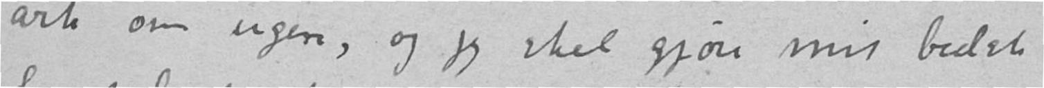ark om ugen, og jeg skal gjøre mit bedste
